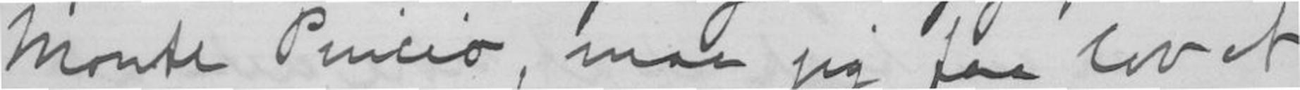Monte Pincio, maa jeg faa lov at
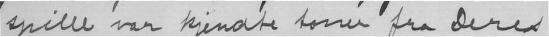spille var kjendte toner fra Deres
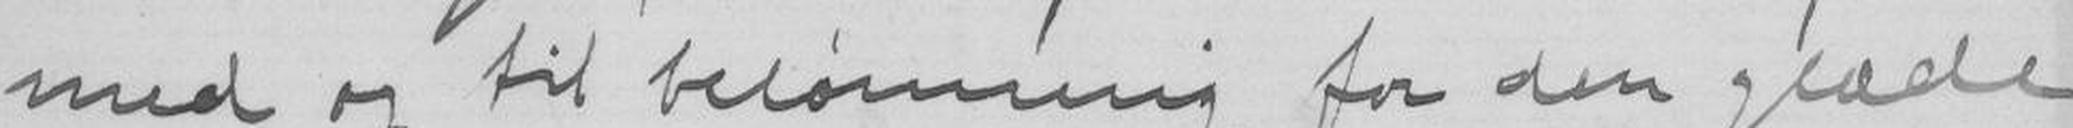med og til belønning for den glæde
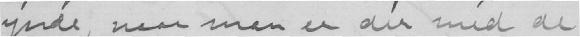ynde, naar man er der med de
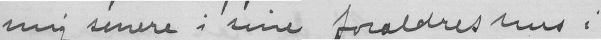mig senere i sine forældres hus i
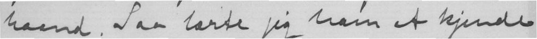haand. Saa lærte jeg ham at kjende
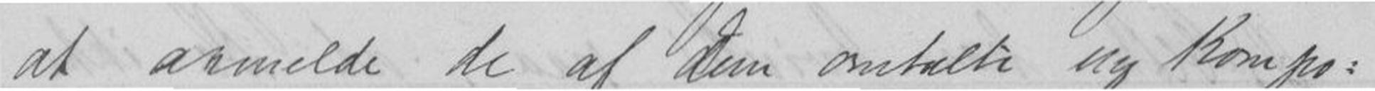at anmelde de af Dem omtalte ny Kompo-
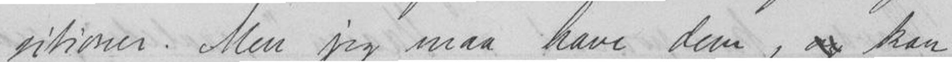sitionen. Men jeg maa have dem, og kan
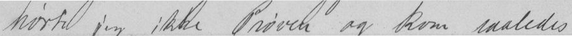hørte jeg ikke Prøven og kom saaledes
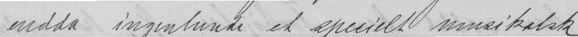endda ingenlunde et spesielt musikalsk
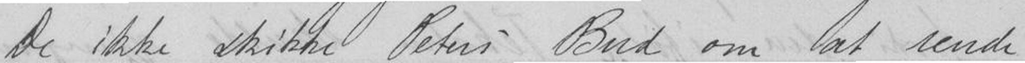De ikke skikke Peters Bud om at sende
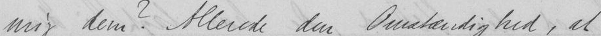mig dem? Allerede den Omstændighed, at
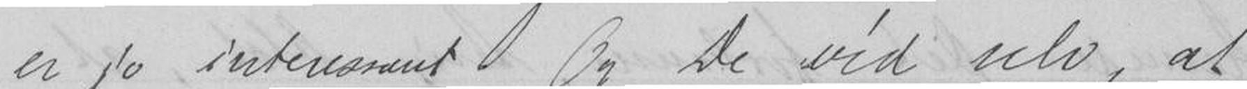er jo interessant. Og De véd selv, at
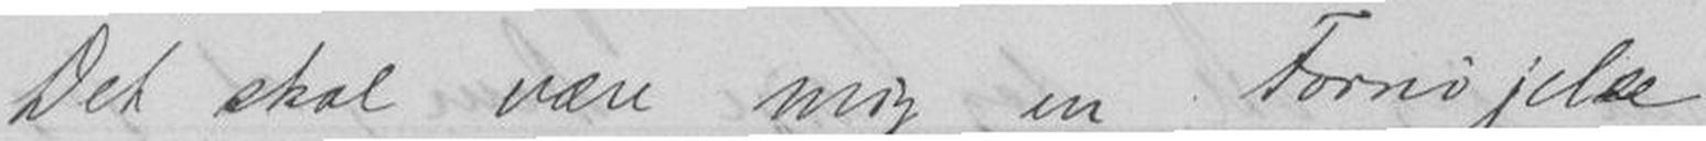Det skal være mig en Fornøjelse
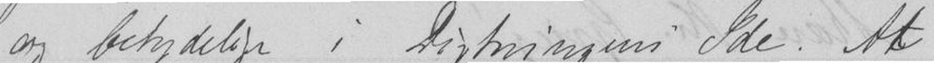og betydelige i Digtningens Ide. At
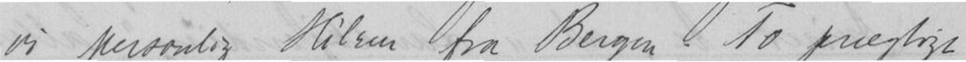vi personlig Hilsen fra Bergen. To pregtige
